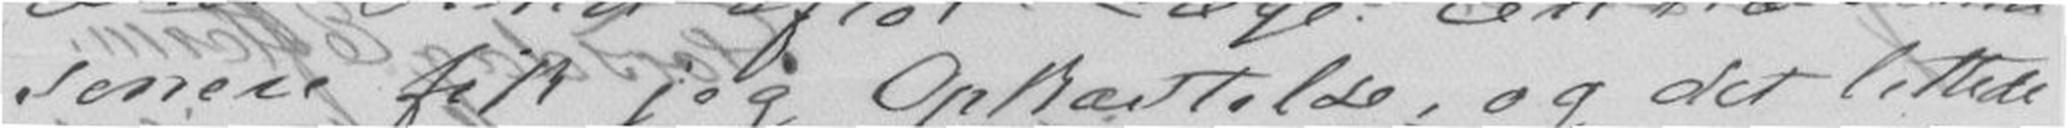senere fik jeg Opkastelse, og det lettede
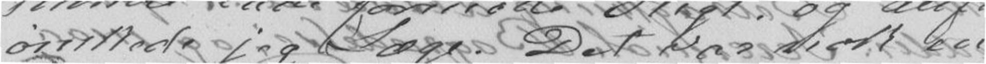ønskede jeg Læge. Det var nok en.
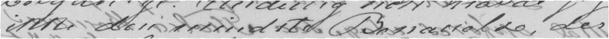ikke den mindste Benanelse, der
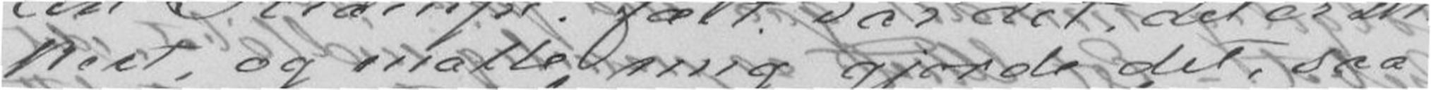kert, og matte mig gjorde det, saa
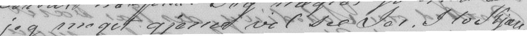jeg meget gjerne vil see Jer. I to Kjære
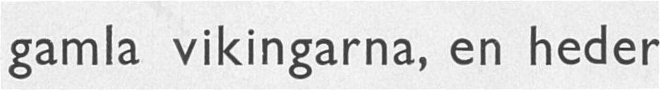gamla vikingarna, en heder
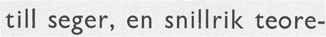till seger, en snillrik teore-
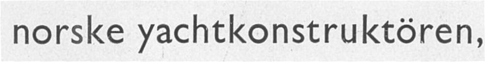norske yachtkonstruktören,
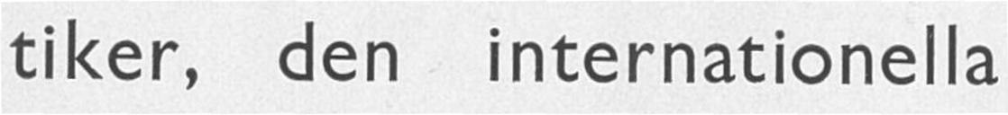tiker, den internationella
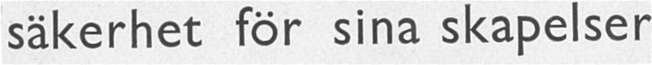säkerhet för sina skapelser
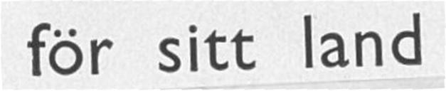för sitt land
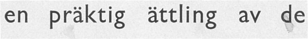en präktig ättling av de
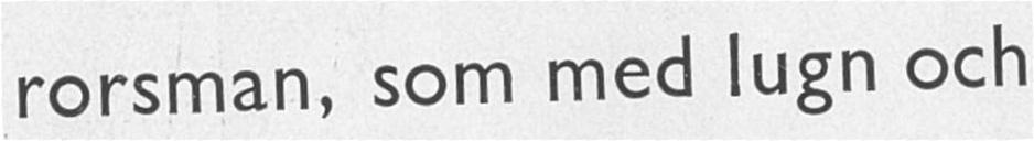rorsman, som med lugn och
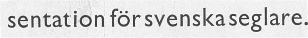sentation för svenska seglare.
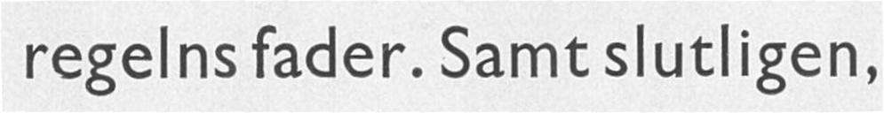regelns fader. Samt slutligen,
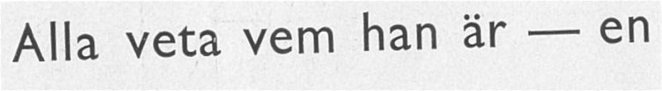Alla veta vem han är — en
